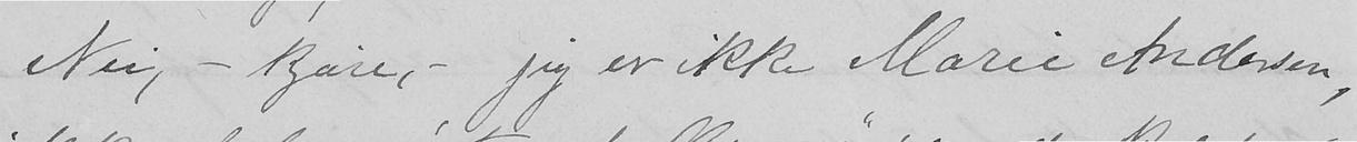Nei, - kjære, - jeg er ikke Marie Andersen,
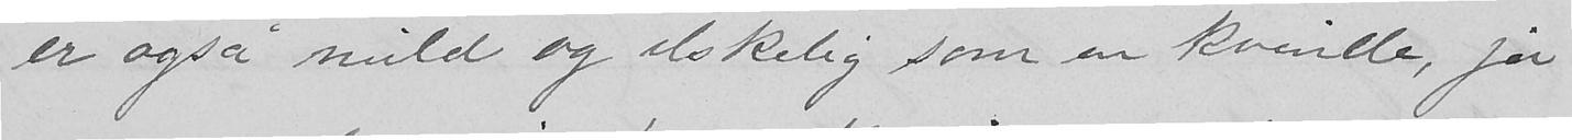er også mild og elskelig som en kvinde, ja
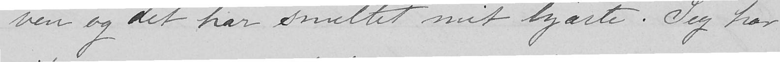ven og det har smeltet mit hjærte. Jeg har
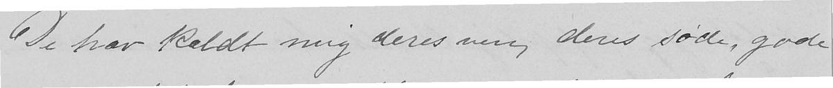De har kaldt mig deres ven, deres søde, gode
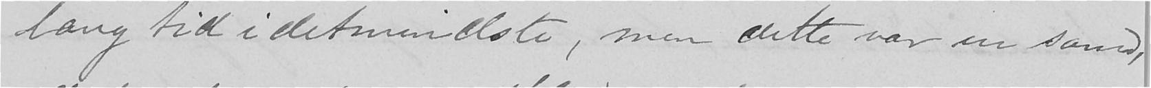lang tid idetmindste, men dette var en sand,
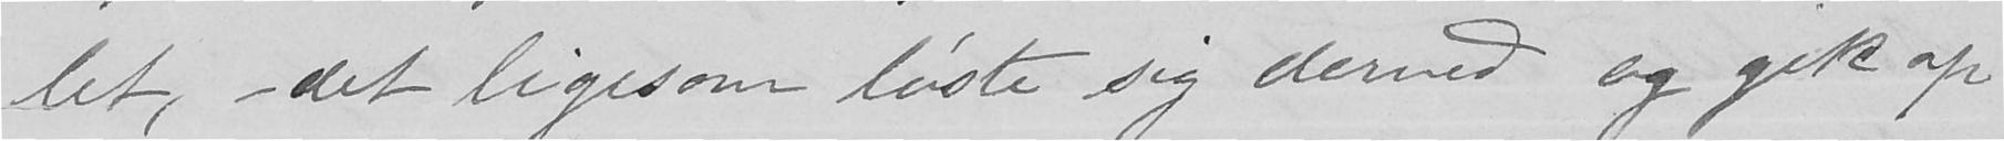let, - det ligesom løste sig derved og gik op
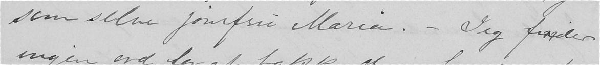som selve jomfru Maria. - Jeg finder
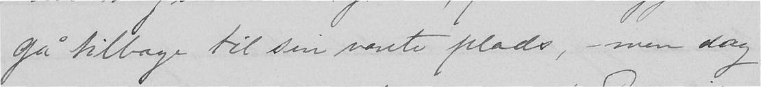gå tilbage til sin vante plads, - men dog
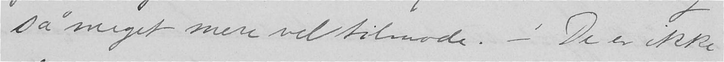så meget mere vel tilmode. - De er ikke
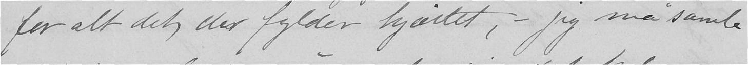for alt det, der fylder hjærtet, - jeg må samle
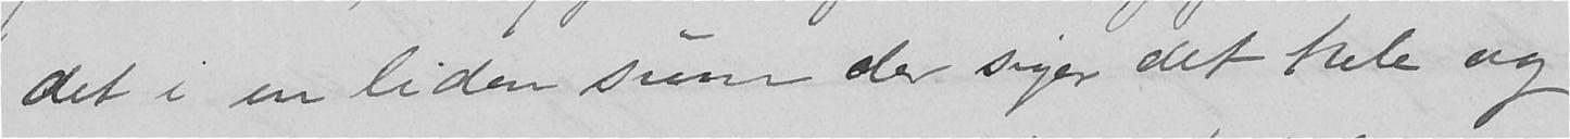det i en liden sum der siger det hele og
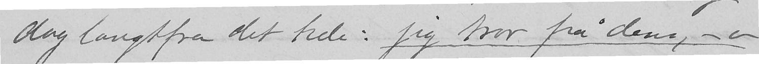dog langtfra det hele: jeg tror på dem, -
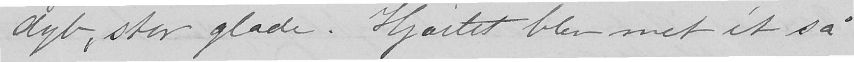dyb, stor glæde. Hjærtet blev met ét så
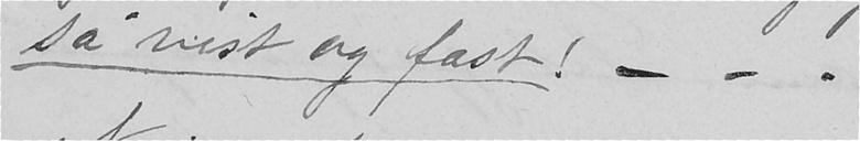så vist og fast! - - -
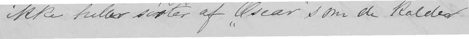ikke heller søster af "Oscar" som de kalder
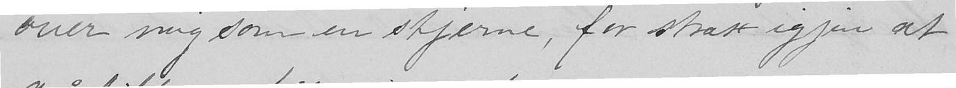over mig som en stjerne, for strakt igjen at
